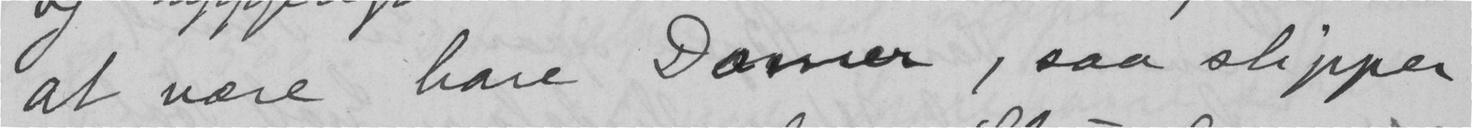at være bare Damer, saa slipper
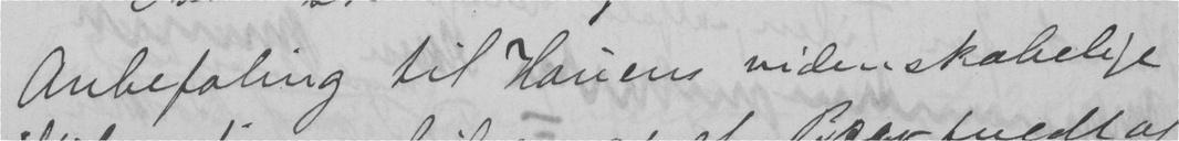Anbefaling til Houens videnskabelige
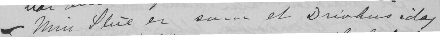- Min Stue er som et Drivhus idag
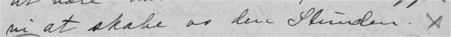vi at skabe os den Stunden. x
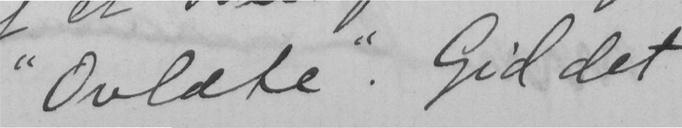"Ovlate". Gid det
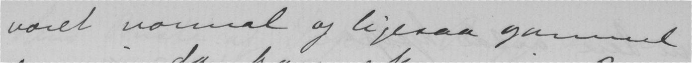været normal og ligesaa gammel
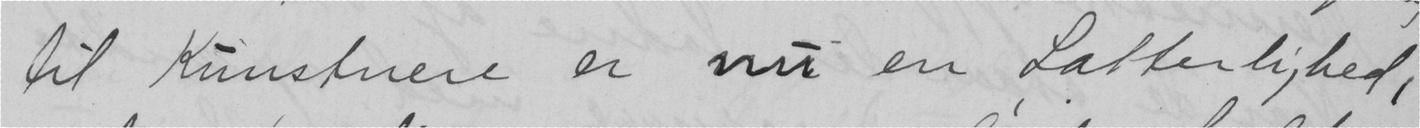til Kunstnere er nu en Latterlighed,
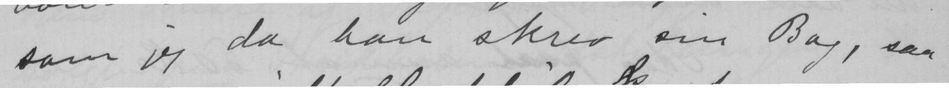som jeg da han skrev sin Bog, saa
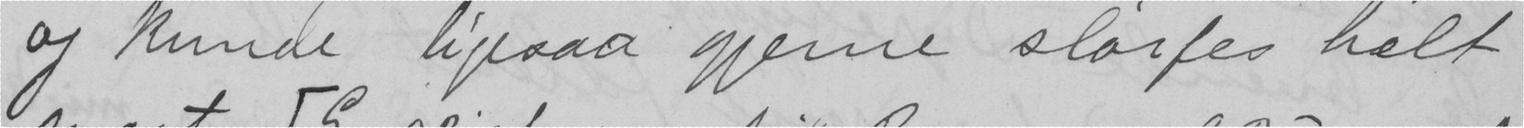og kunde ligesaa gjerne sløifes helt
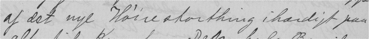af det nye Høirestorthing ihærdigt paa
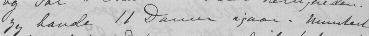Jeg havde 11 Damer igaar. Muntert
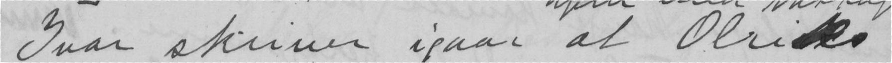Ivar skriver igaar at Olriks
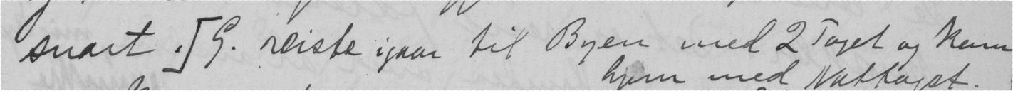snart. G. reiste igaar til Byen med 2 Toget og kom
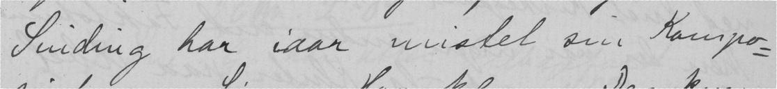Sinding har iaar mistet sin Kompo-
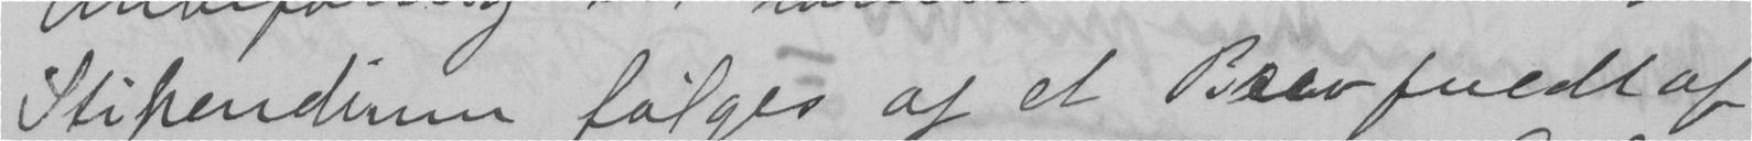Stipendium følges af et Brev fuldt af
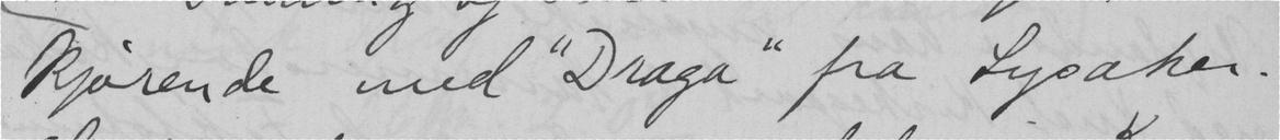kjørende med "Draga" fra Lysaker.
